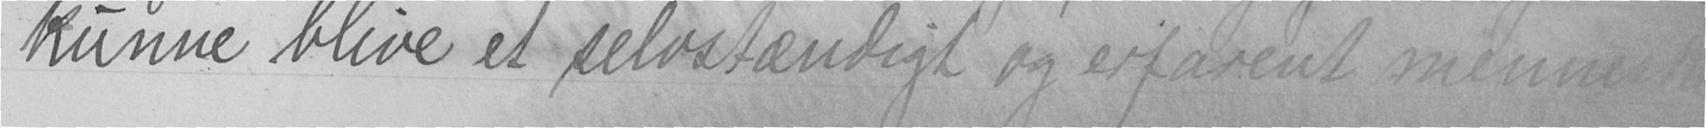kunne blive et selvstændigt og erfarent menneske
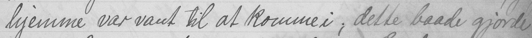hjemme var vant til at komme i; dette baade gjorde
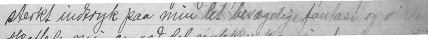sterkt indtryk paa min let bevægelige fantasi og sikkert
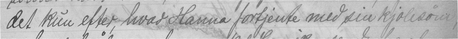det kun efter, hvad Hanna fortjente med sin kjolesøm,
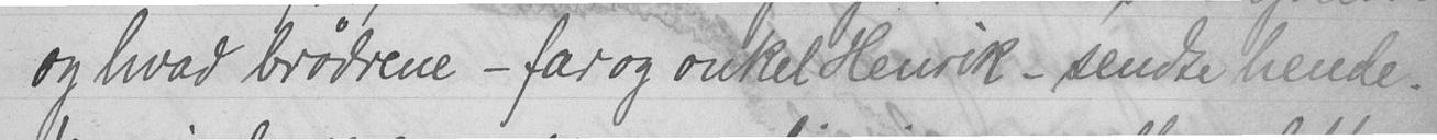og hvad brødrene - far og onkel Henrik - sendte hende.
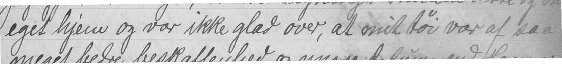eget hjem og var ikke glad over, at mit tøi var af saa
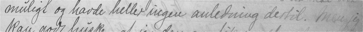muligt og havde heller ingen anledning dertil. Men jeg
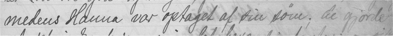medens Hanna var optaget af sin søm; de gjorde
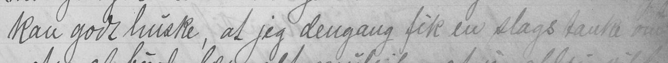kan godt huske, at jeg dengang fik en slags tanke om
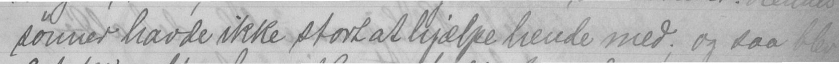sønner havde ikke stort at hjælpe hende med; og saa blev
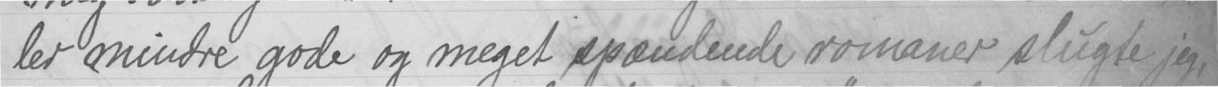ler mindre gode og meget spændende romaner slugte jeg,
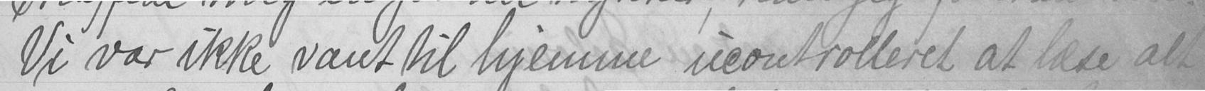Vi var ikke vant til hjemme ucontrolleret at læse alt
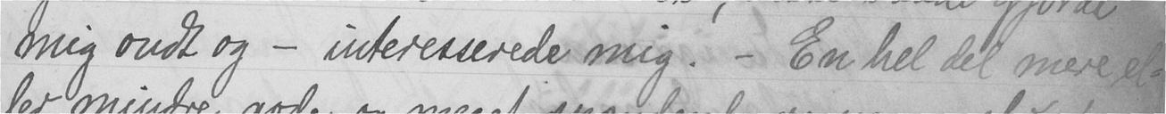mig ondt og - interesserede mig. - En hel del mere el-
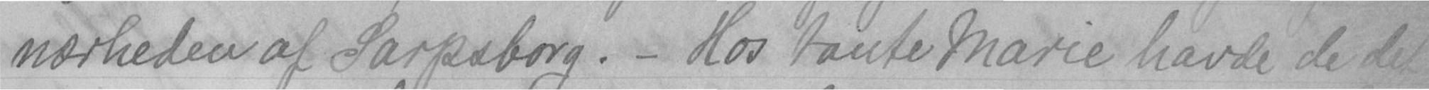nærheden af Sarpsborg. - Hos tante Marie havde de det
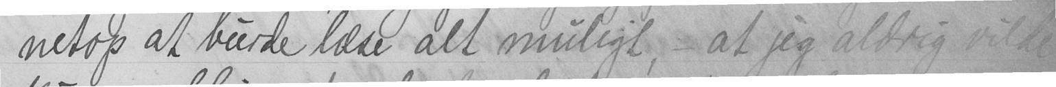netop at burde læse alt muligt, - at jeg aldrig vilde
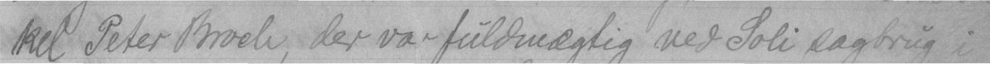kel Peter Broch, der var fuldmægtig ved Soli sagbrug i
